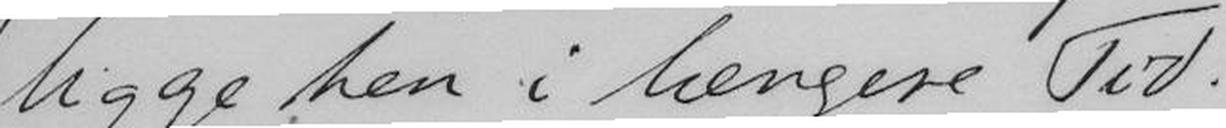ligge her i lengere Tid.
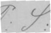P:S:
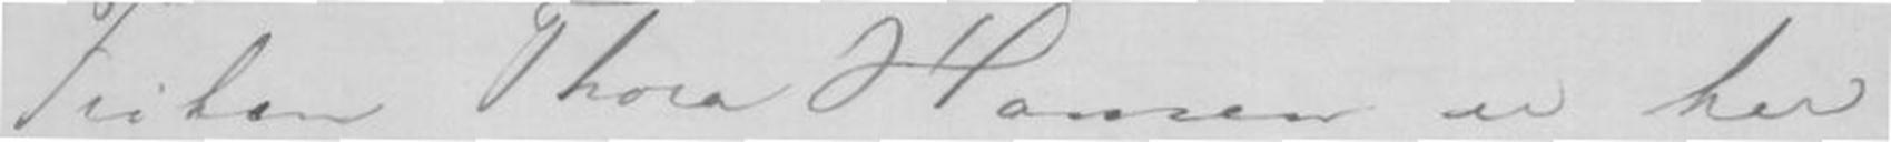Frøken Thora Hansen er her
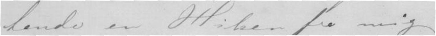hende en Hilsen fra mig
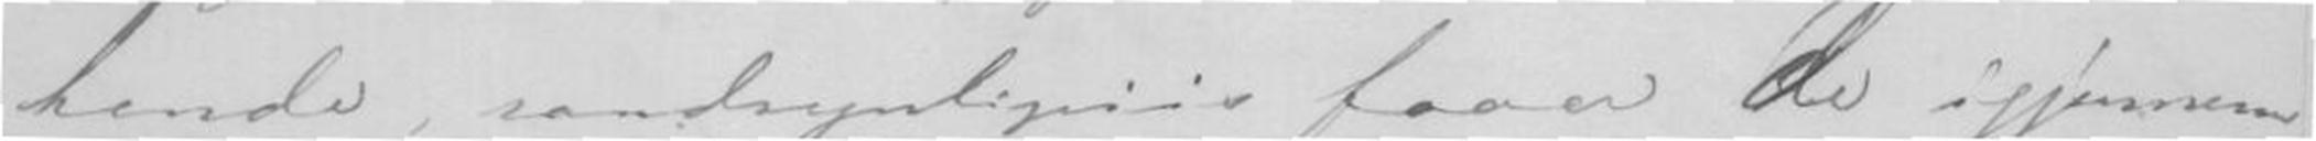hende, sandsynligvis faaer De igjennem
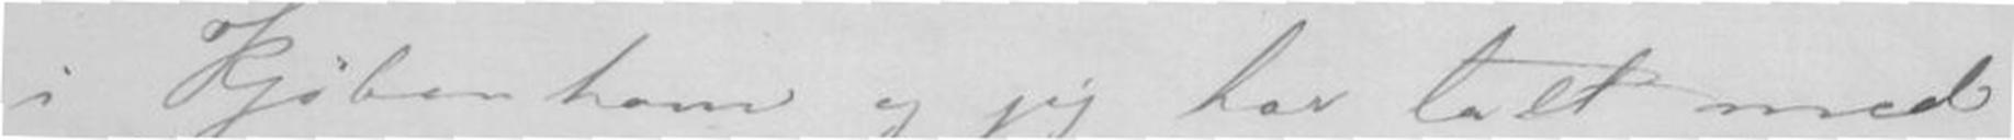i Kjøbenhavn og jeg har talt med
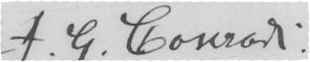J.G. Conradi.
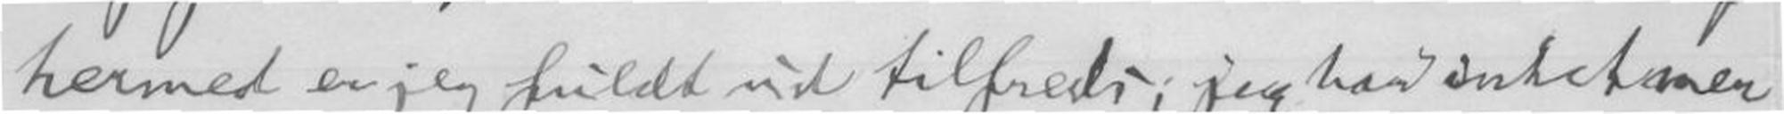hermed er jeg fuldt tilfreds; jeg har intet mer
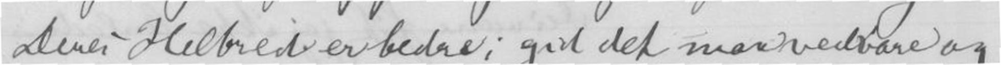Deres Helbred er bedre; gid det maa vedvare og
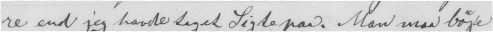re end jeg havde taget Sigte paa. Man maa bøje
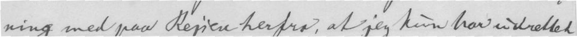ning med paa Rejsen herfra, at jeg kun har udrettet
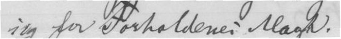sig for Forholdernes Magt.
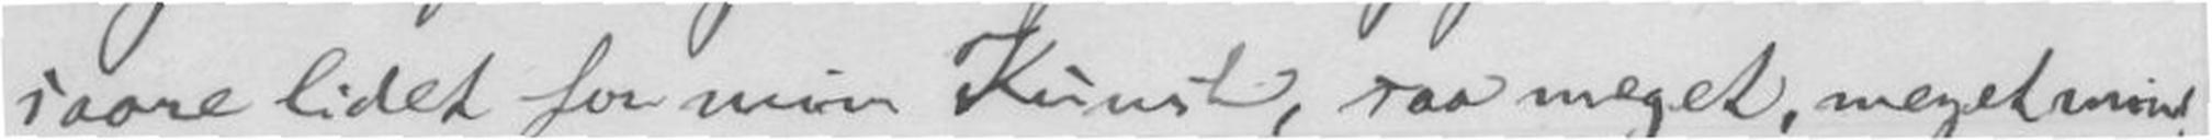saare lidet for min Kunst, saa meget, meget mind-
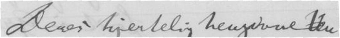Deres hjertelig hengivne Ven
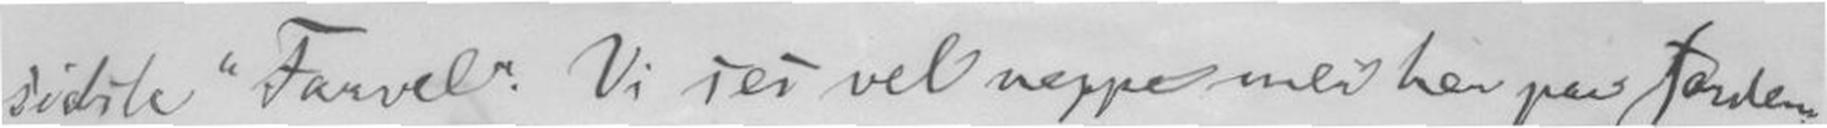sidste "Farvel". Vi ser vel neppe mer her paa Jorden,
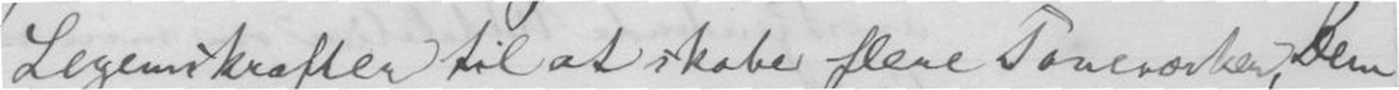Legemskræfter til at skabe flere Toneværker, Dem
